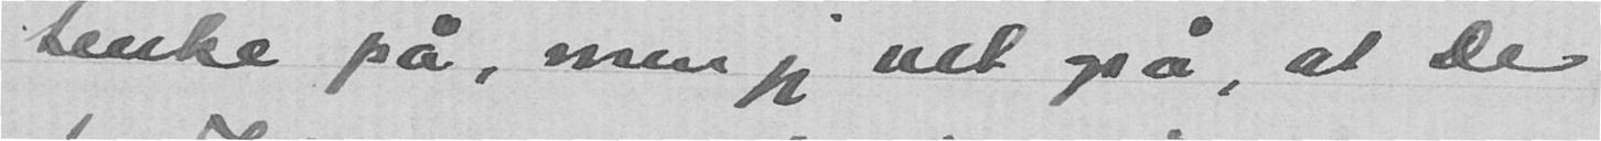tenke på, men jeg vet også, at De
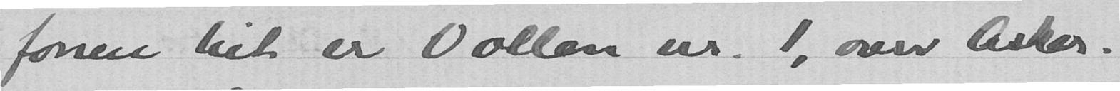fonen hit er Vollen nr 1, over Asker.
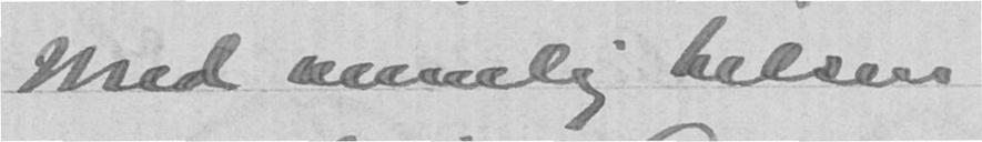Med venlig hilsen
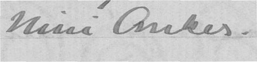Nini Anker
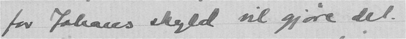for Johans skyld vil gjøre det .
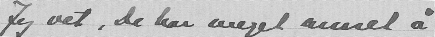Jeg vet, de har meget annet å
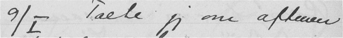9/I Talte jeg om aftenen
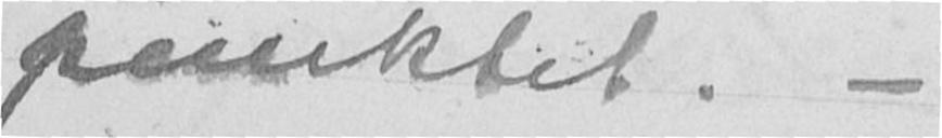punktet. -
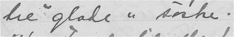tre "glade" søstre.
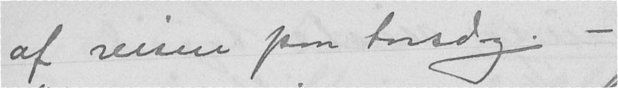af reisen paa torsdag. -
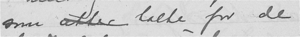som atter talte for de
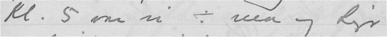Kl. 5 var vi ÷ ma og Sigr
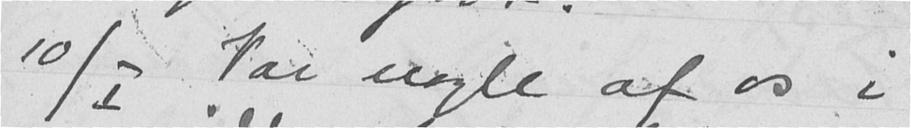10/I Var nogle af os i
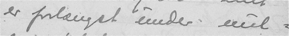er forlængst under nul-
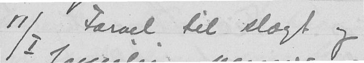11/I Farvel til slægt og
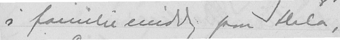i familiemiddag paa Hola,
